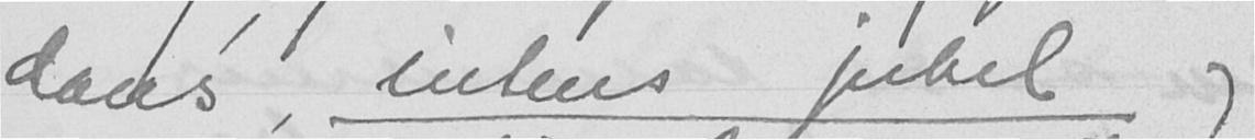dans, intens jubel og
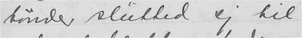bønder slutted sig til
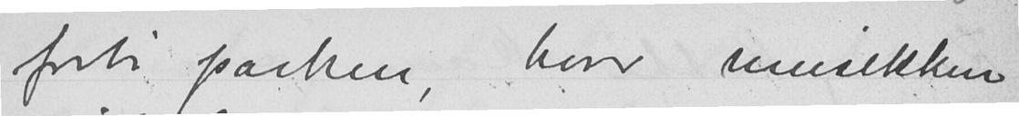forbi parken, hvor musikken
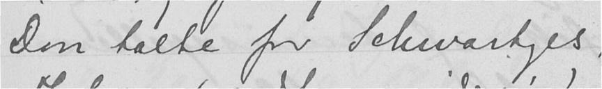Don talte for Schwartzes,
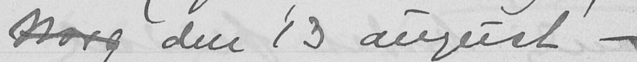Norg den 13 august -
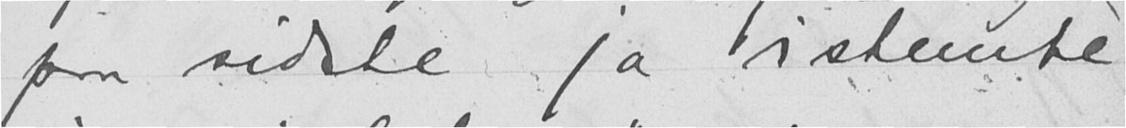paa sidste ja istemte
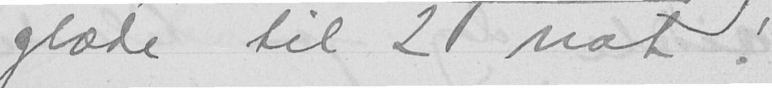glæde til 2 nat!
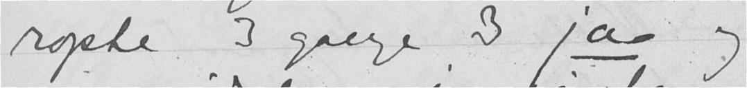ropte 3 gange 3 ja og
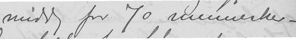middag for 70 mennesker -
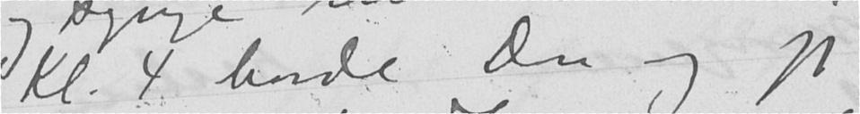Kl. 4 havde Don og jeg
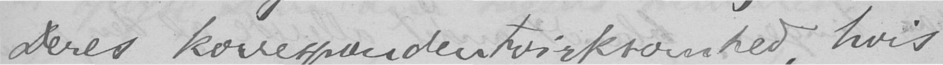Deres korrespondentvirksomhed, hvis
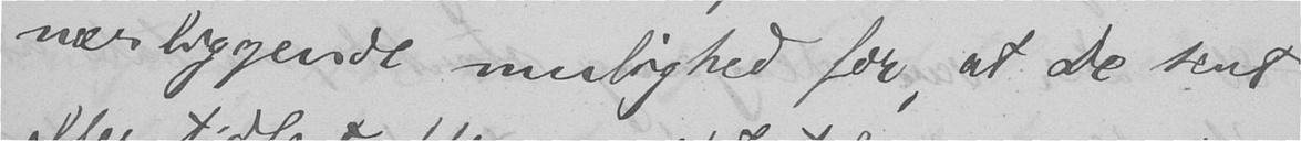nærliggende mulighed for, at De sent
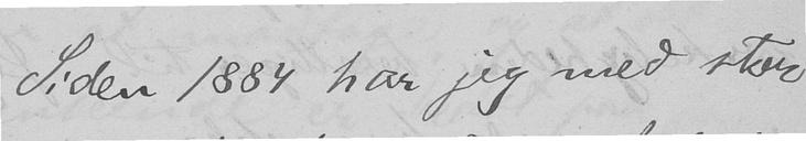Siden 1884 har jeg med stor
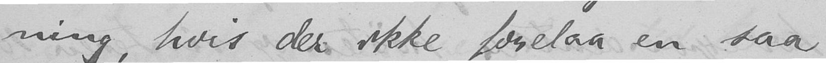ning, hvis der ikke forelaa en saa
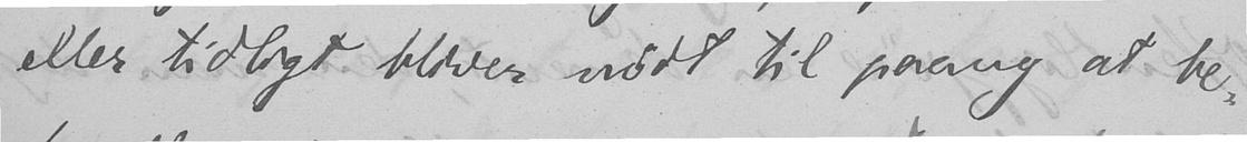eller tidligt bliver nødt til paany at be-
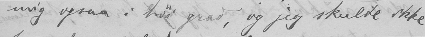mig ogsaa i høi grad, og jeg skulde ikke
